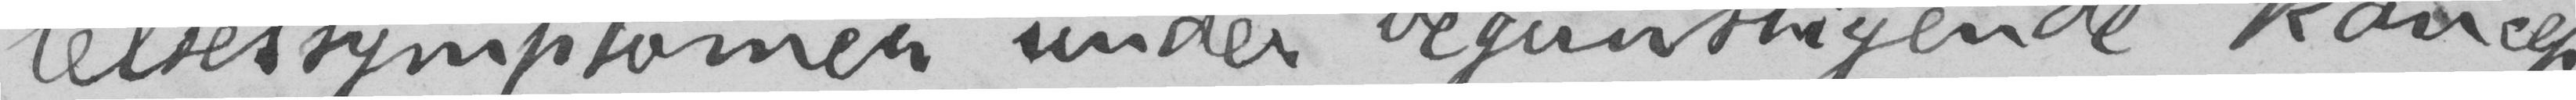lelsessymptomer under begunstigende koncep-
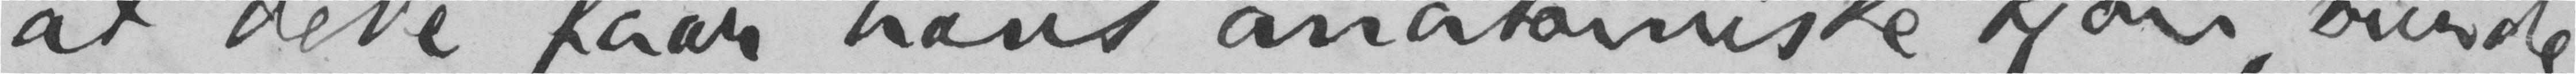at dette faar hans anatomiske kjøn, burde
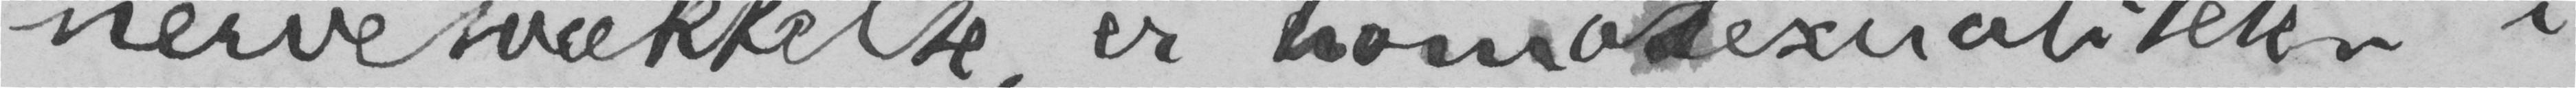nervesvækkelse, er homosexualiteten i
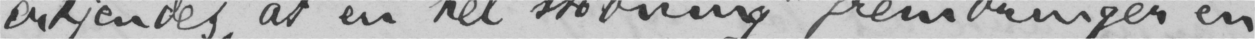erkjendes, at «en hel støbning» frembringer en
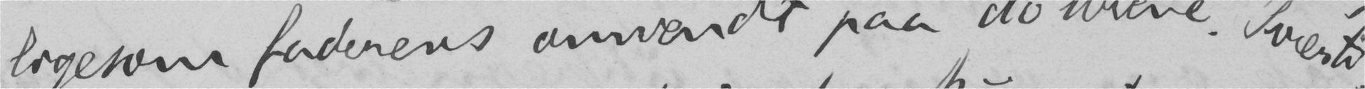ligesom faderens omvendt paa døttrene. Tværti-
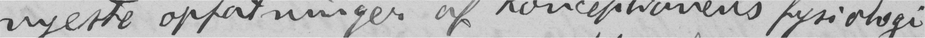nyeste opfatninger af konceptionens fysiologi
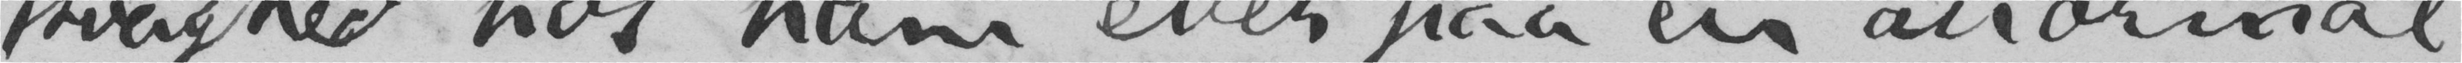svaghed hos ham eller paa en anormal
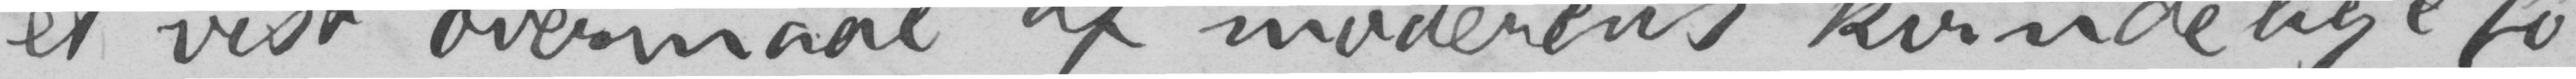et vist overmaal af moderens kvindelige fø-
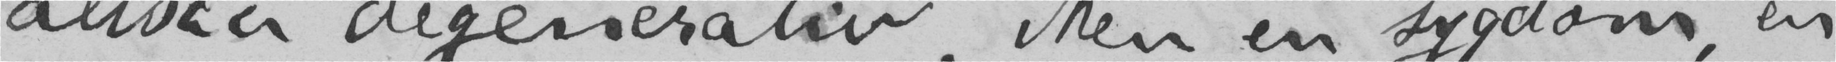altsaa degenerativ. Men en sygdom, en
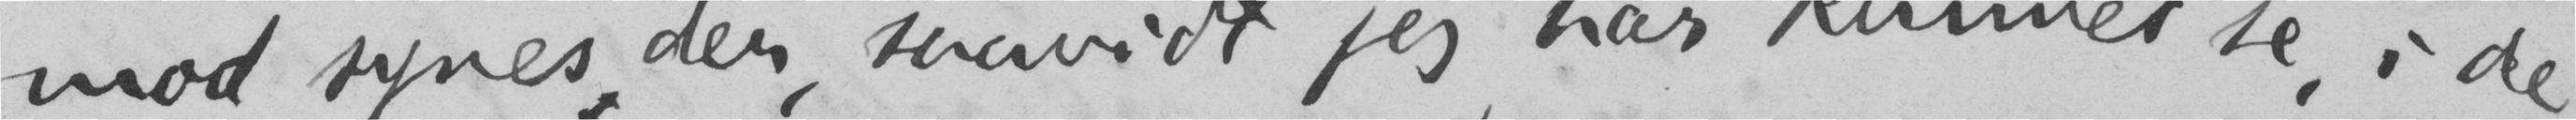mod synes der, saavidt jeg har kunnet se, i de
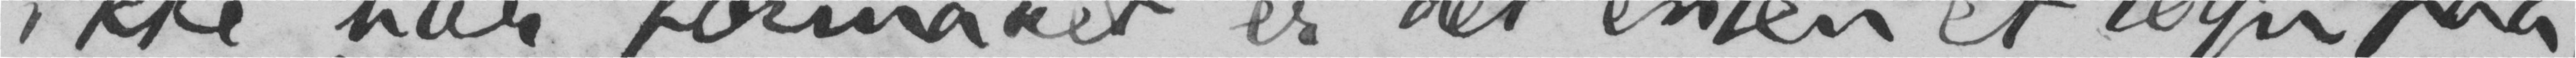ikke har formaaet, er det enten et tegn paa
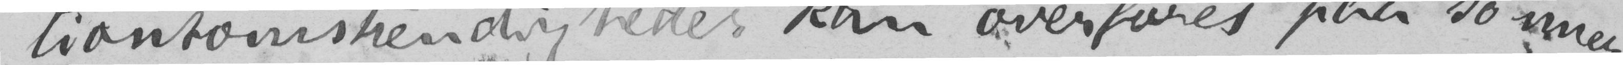tionsomstændigheder kan overføres paa sønnerne
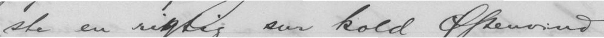ste en rigtig sur kold Østenvind
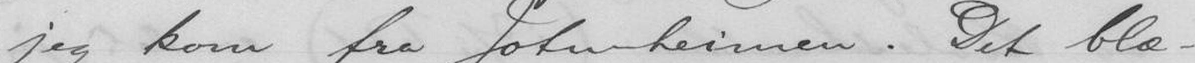jeg kom fra Jotunheimen. Det blæ-
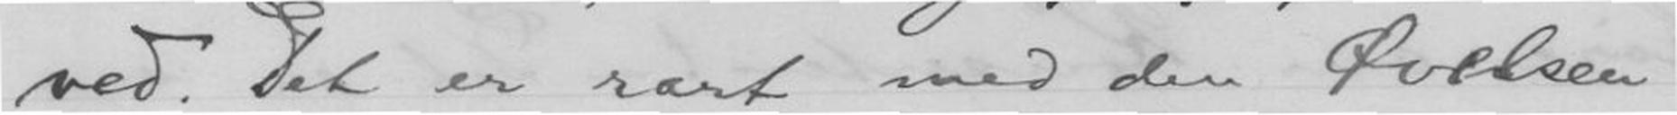ved. Det er rart med den Øvelsen,
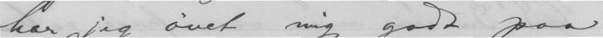har jeg øvet mig godt paa
